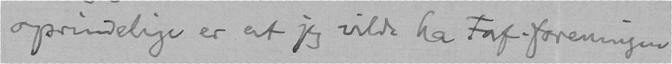oprindelige er at jeg vilde ha Forf.foreningen
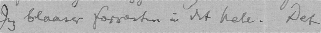Jeg blaaser forresten i det hele. Det
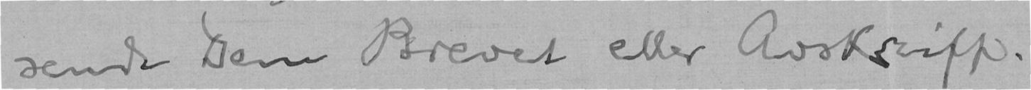sende Dem Brevet eller Avskrift.
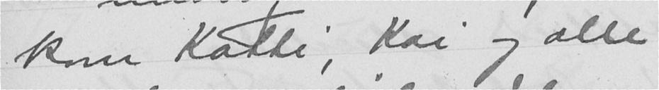kom Katti, Kai og alle
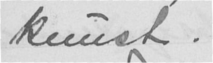knust.
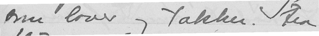som lover og takker. Fra
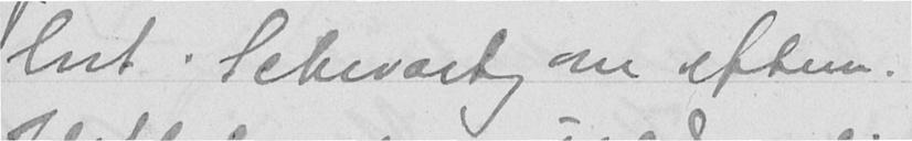lnt. Schwartz om efterm.
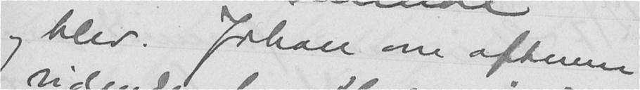og blev. Johan om aftenen
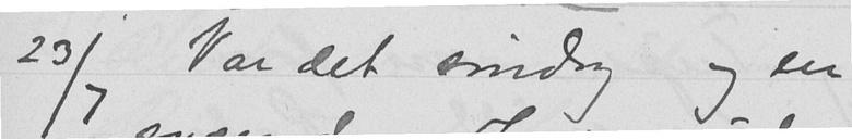23/7 Var det søndag og en
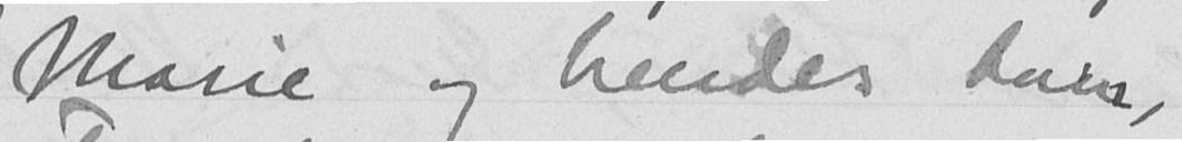Marie og hendes barn,
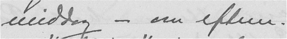middag - om efterm.
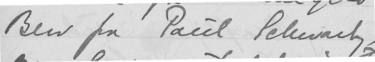Brev fra Paul Schwartz,
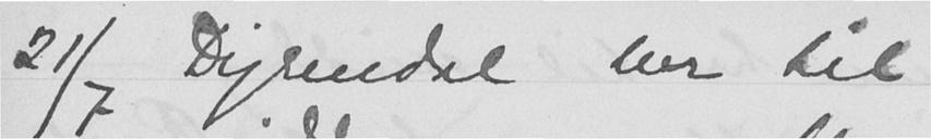21/7 Dyrendal her til
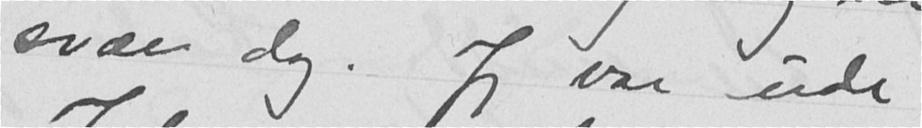svær dag. Jeg var ude
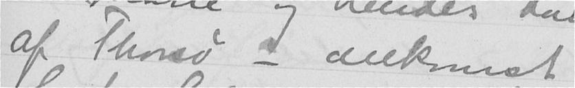af Thorsø - ankomst
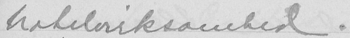hotelvirksomhed.
